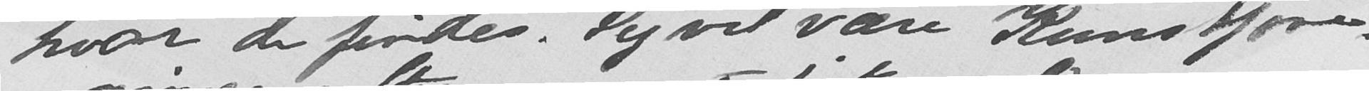hvor de findes. Jeg vil være Kunstfore-
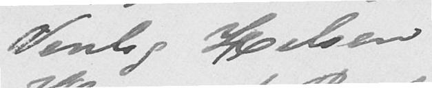Venlig Hilsen
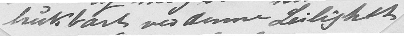brukbart ved denne Leilighet.
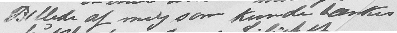Billede af mig som kunde tænkes
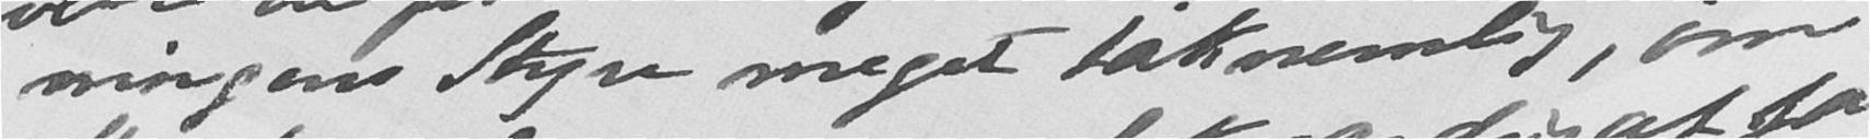ningens Styre meget taknemlig, om
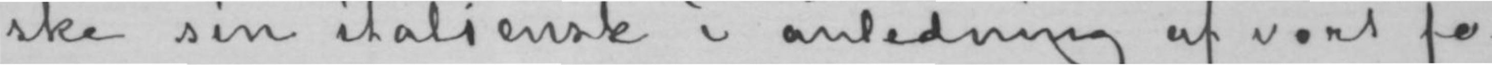ske sin italiensk i anledning af vort fo-
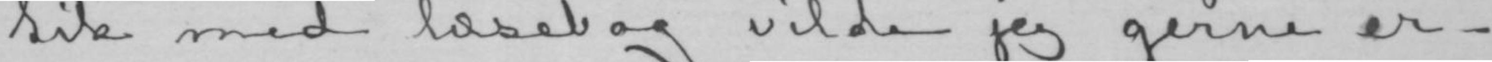tik med læsebog vilde jeg gerne er-
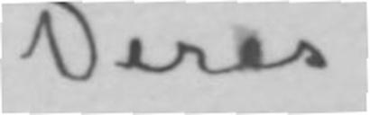Deres
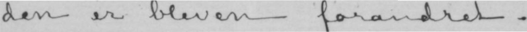den er bleven forandret.
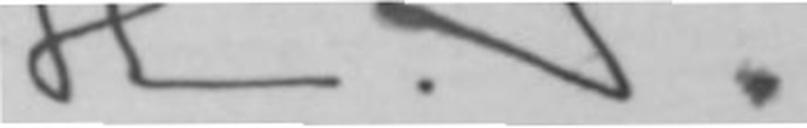H. I.
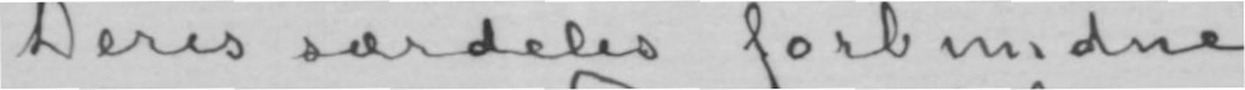Deres særdeles forbundne
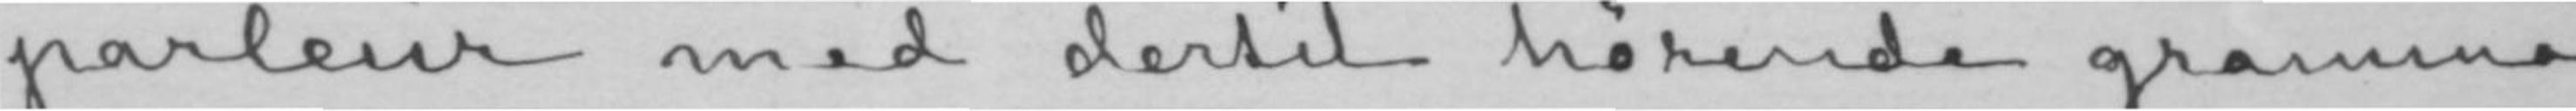parleur med dertil hørende gramma-
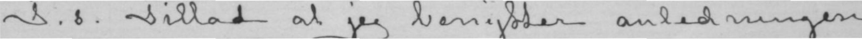P. s. Tillad at jeg benytter anledningen
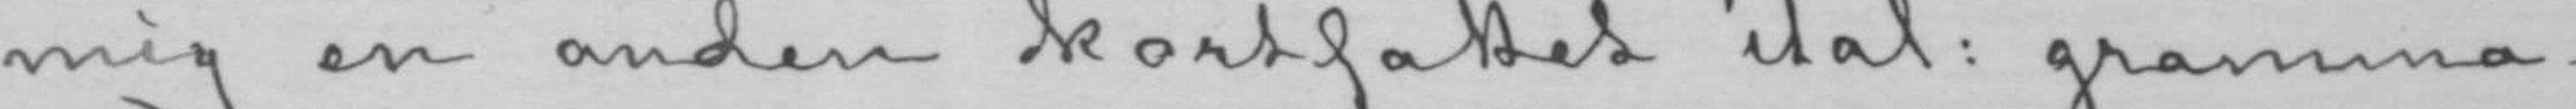mig en anden kortfattet ital: gramma-
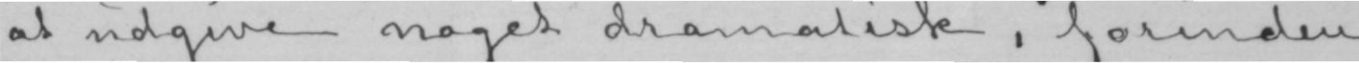at udgive noget dramatisk, forinden
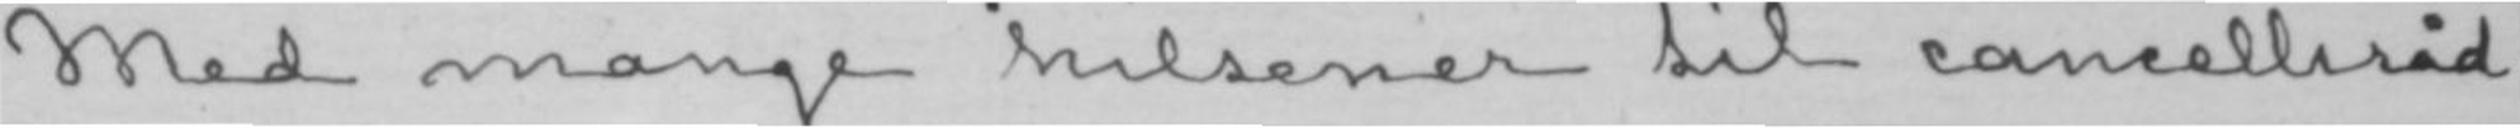Med mange hilsener til cancelliråd
